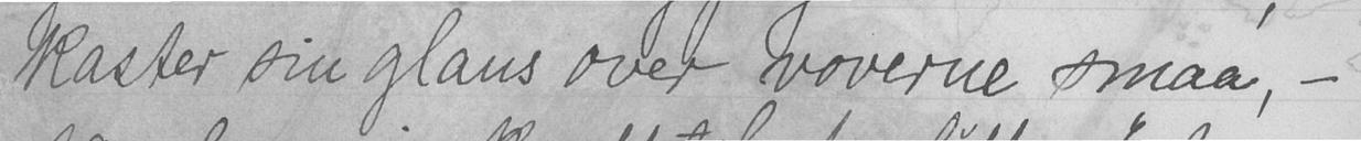kaster sin glans over voverne smaa, -
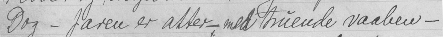Dog - faren er atter - med truende vaaben -
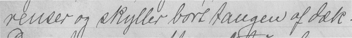renser og skyller bort tangen af dæk.
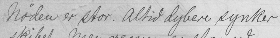Nøden er stor. Altid dybere synker
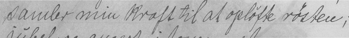samler min kraft til at opløfte røsten;
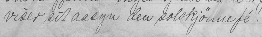viser sit aasyn den solskjønne fé.
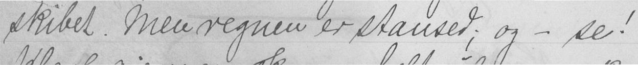skibet. Men regnen er stansed; og - se!
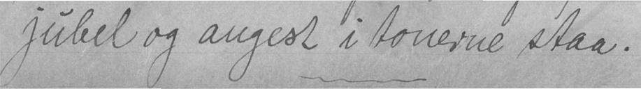jubel og angest i tonerne staa.
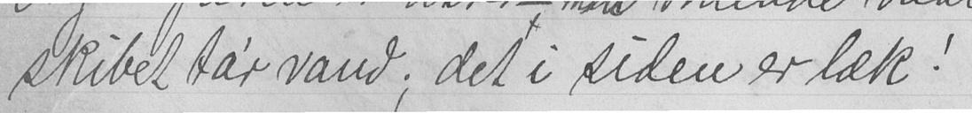skibet ta'r vand; det i siden er læk!
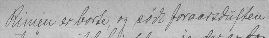Rimen er borte, og sødt foraarsduften
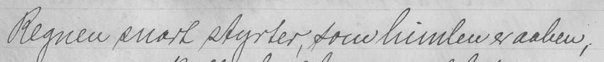Regnen snart styrter, som himlen er aaben,
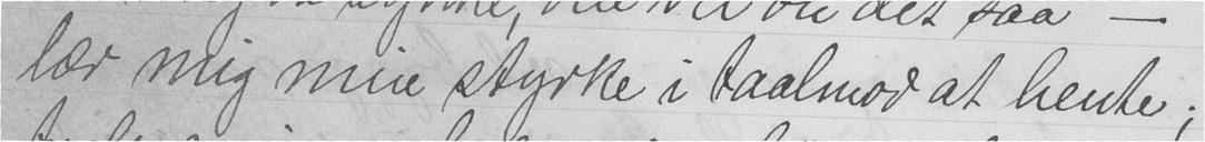lær mig min styrke i taalmod at hente;
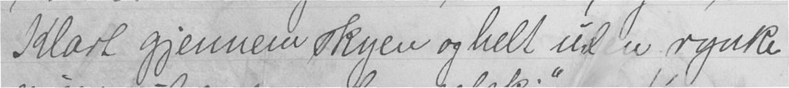Klart gjennem skyen og helt uden rynke
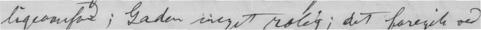ligeoverfor; Gaden meget rolig; det foregik ved
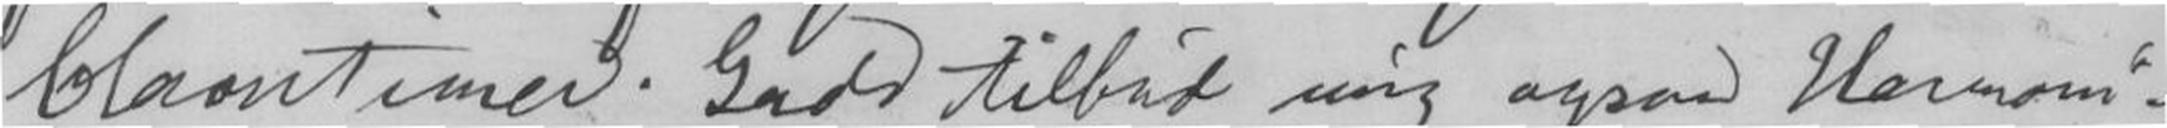Clavertimer. Gade tilbød mig ogsaa Harmoni-
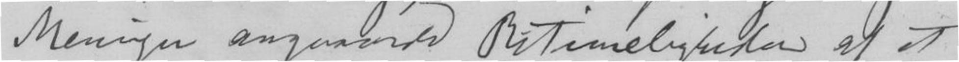Meninger angaaende Betimeligheden af at
